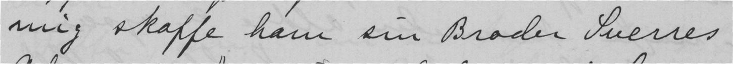mig skaffe ham sin Broder Sverres
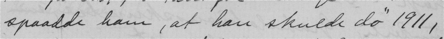spaadde ham, at han skulde do" 1911,
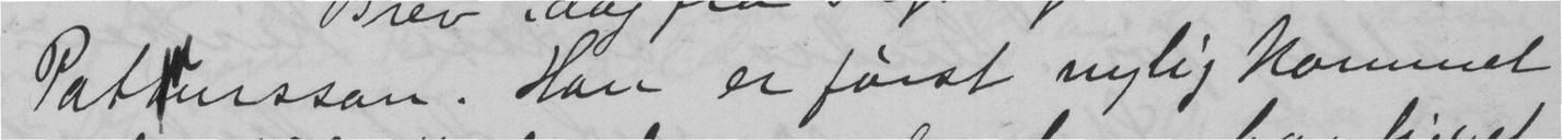Patursson. Han er først nylig kommet
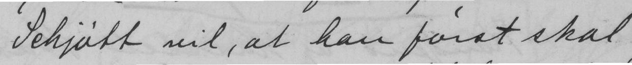Schjøtt vil, at han først skal
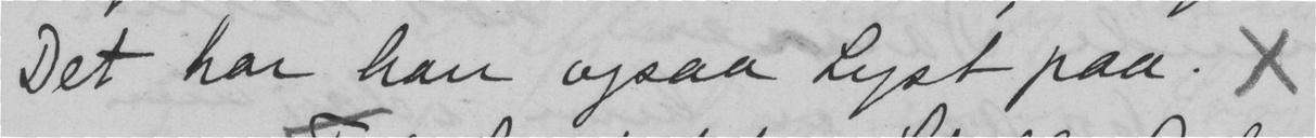Det har han ogsaa Lyst paa. x
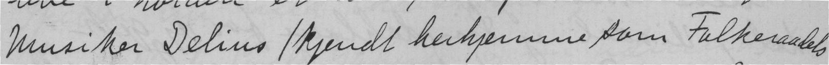musiker Delius (kjendt herhjemme som Folkeraadets
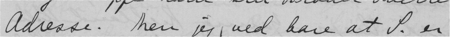adresse. Men jeg ved bare at S. er
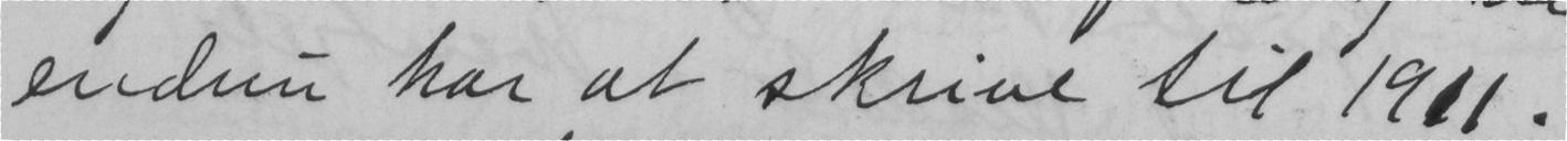endnu har at skrive til 1911.
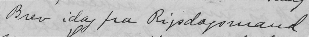Brev idag fra Rigsdagsmand
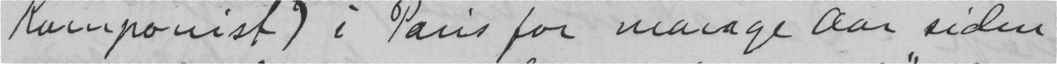Komponist) i Paris for mange aar siden
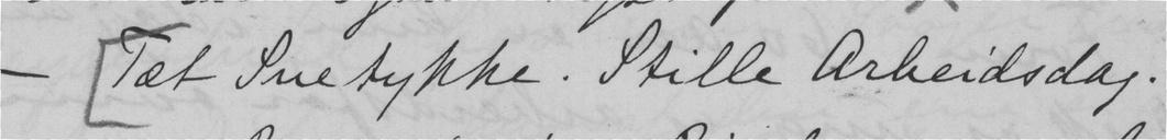- Tæt Snetykke. Stille arbeidsdag.
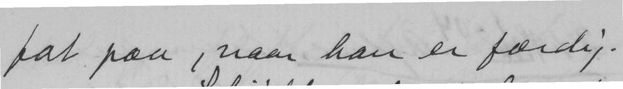fat paa, naar han er færdig.
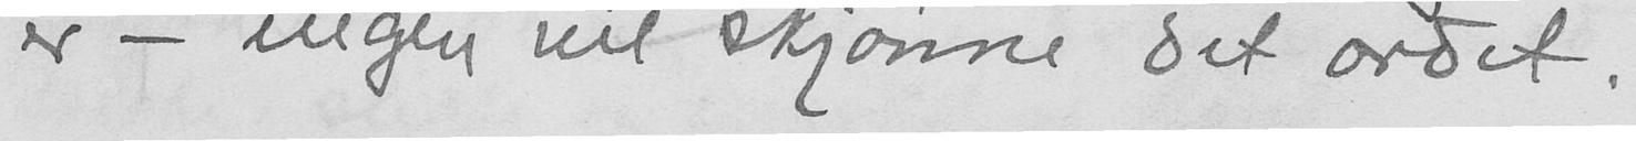er - ingen vil skjønne det ordet.
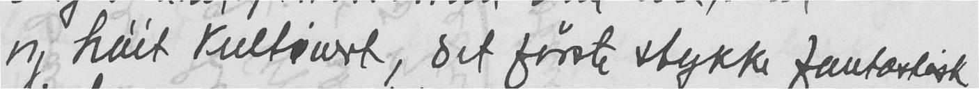og høit kultivert, det første stykke fantastisk,
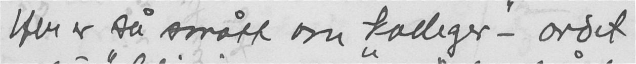Her er så smått om kolleger - ordet
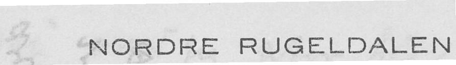NORDRE RUGELDALEN
